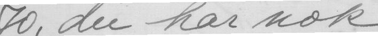Jo, du har nok
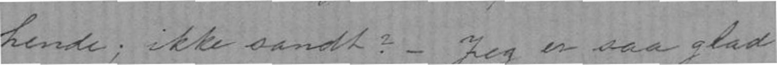hende; ikke sandt? - Jeg er saa glad
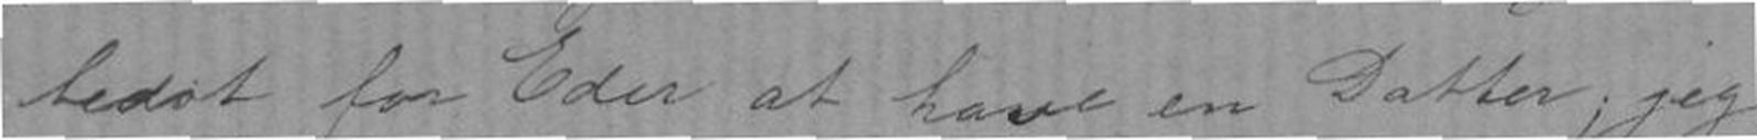bedst for Eder at have en Datter; jeg
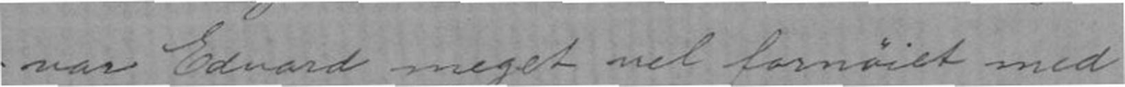var Edvard meget vel fornøiet med
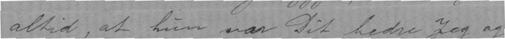altid, at hun var Dit bedre Jeg og
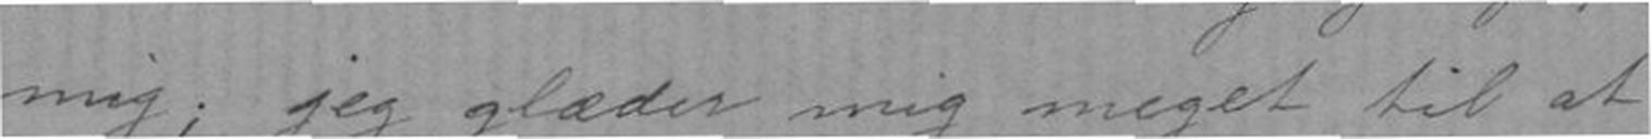mig; jeg glæder mig meget til at
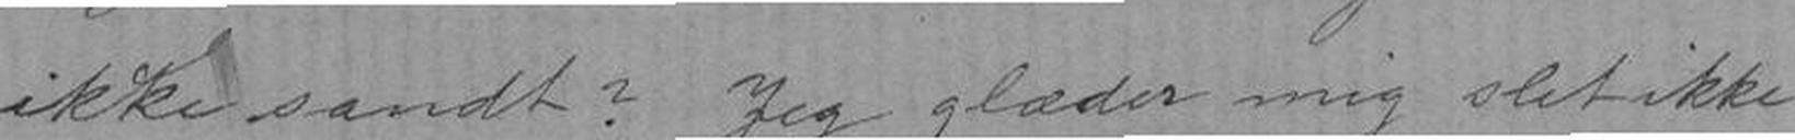ikke sandt? Jeg glæder mig slet ikke
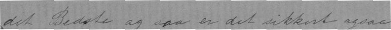det Bedste og saa er det sikkery ogsaa
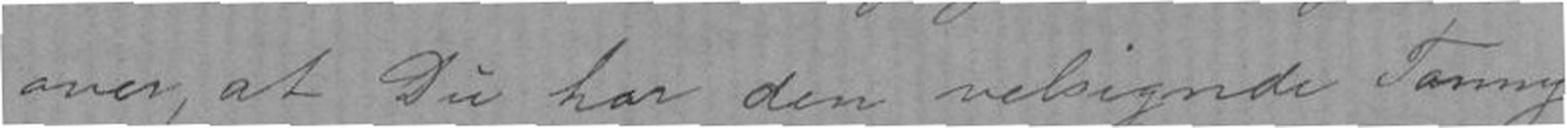over, at Du har den velsignede Tonny
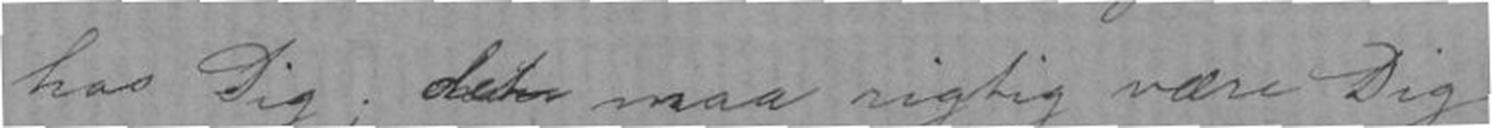hos Dig; det maa rigtig være Dig
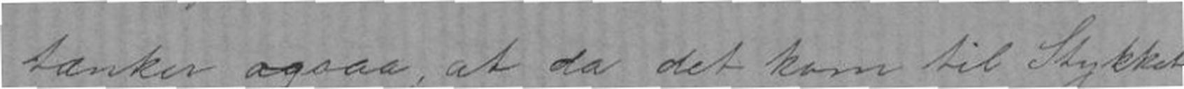tænker ogsaa, at da det kom til Stykket
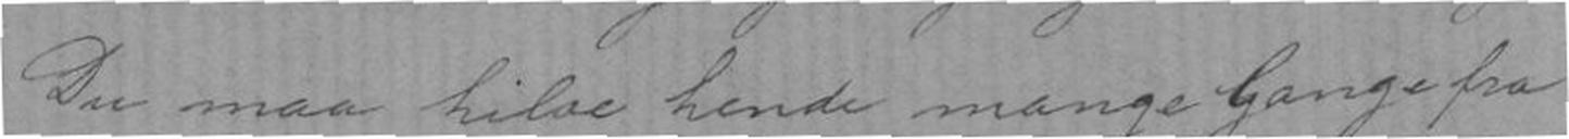Du maa hilse hende mange Gange fra
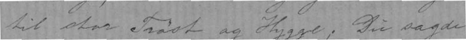til stor Trøst og Hygge; Du sagde
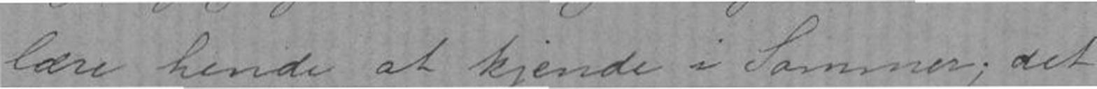lære hende at kjende i Sommer; det
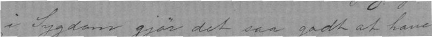i Sygdom gjør det saa godt at have
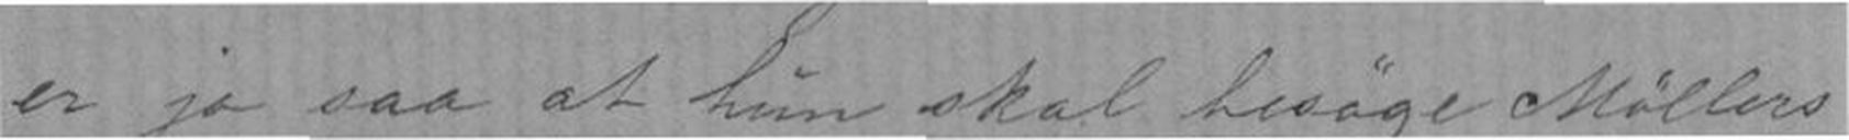er jo saa at hun skal besøge Møllers
